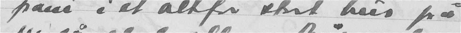pani i et altfor stort hus på
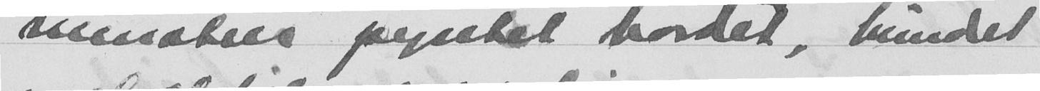minaties pyntet bordet, bundet
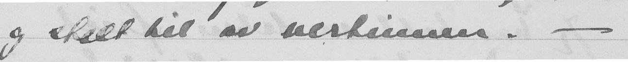og stælt til av nestimen. -
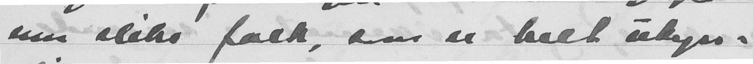enn slike folk, som er helt ukyn-
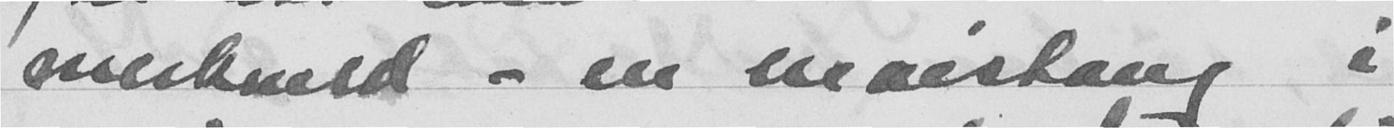merkveld - en maestang i
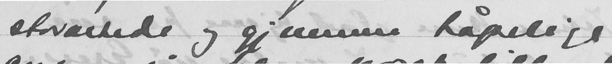storartede og gjennom tåpelige
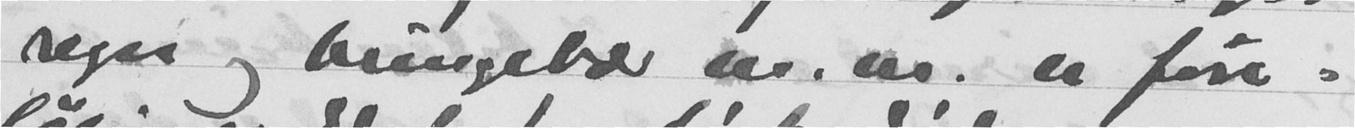regn og bringebær m. m. er får-
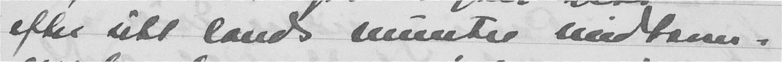efter sitt lands munter midtsom-
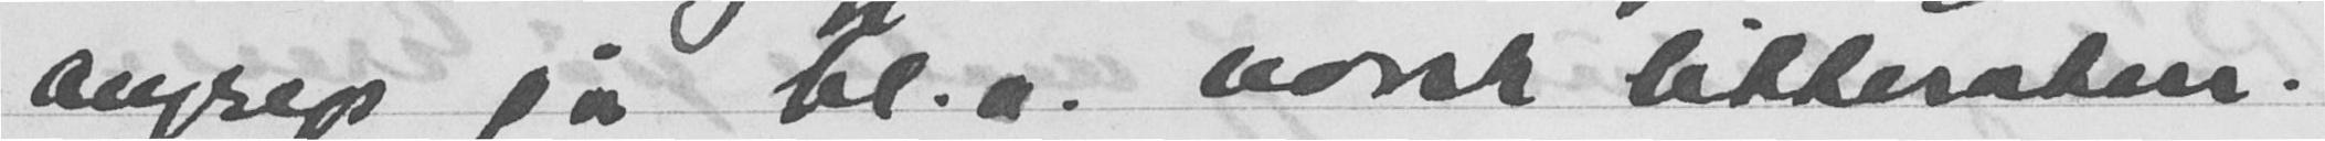angrep på bl. a. norsk litteratur.
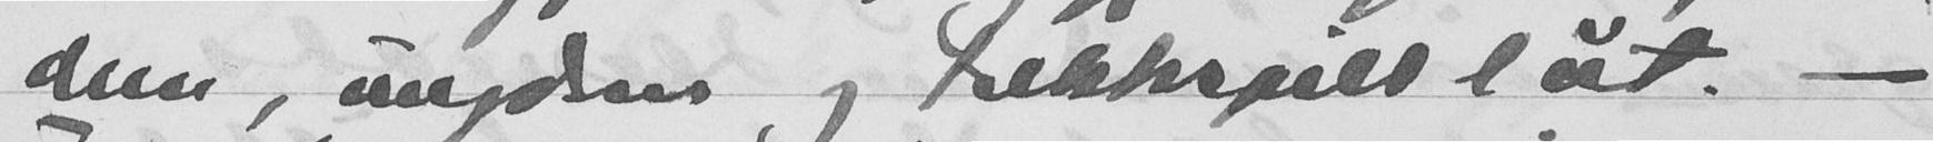dem, ungdom og trekkspil låt. -
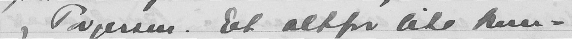og Porgessen. Et altfor lite kom-
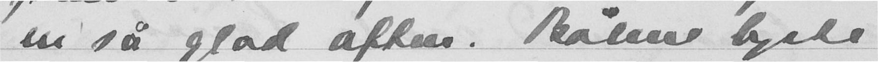en så glad aften. Båten lyste
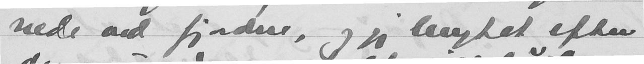nede ved fjorden, og jeg lengtet efter
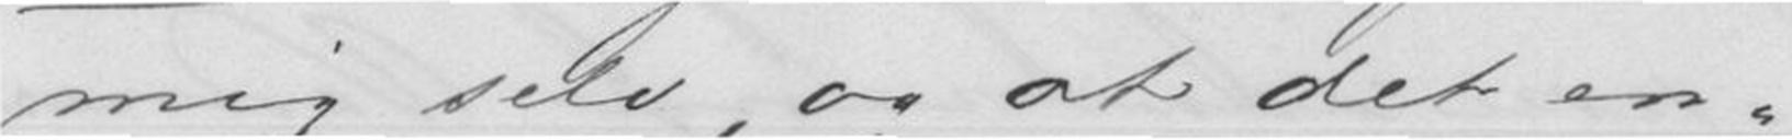mig selv, og at det en-
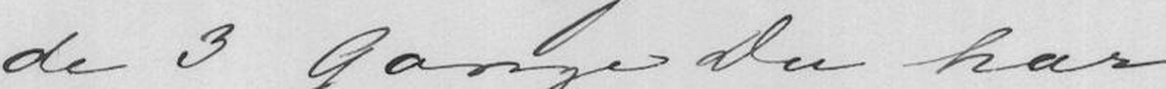de 3 Gange Du har
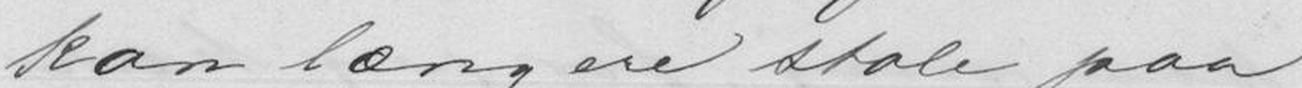kan længere stole paa
